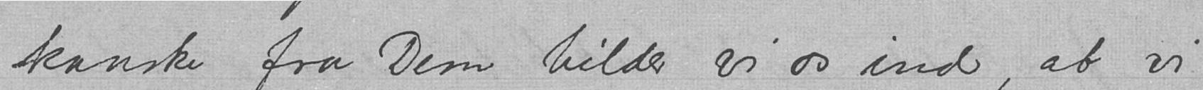kanske fra Dem bilder vi os ind, at vi
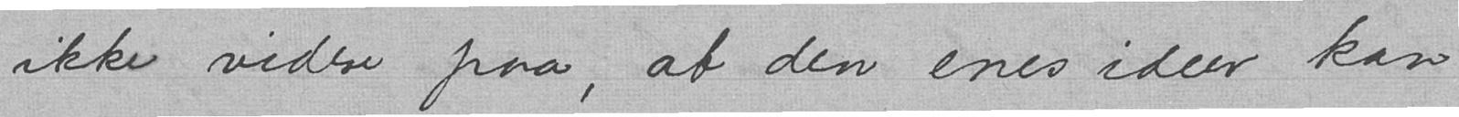ikke videre paa, at den enes ideer kan
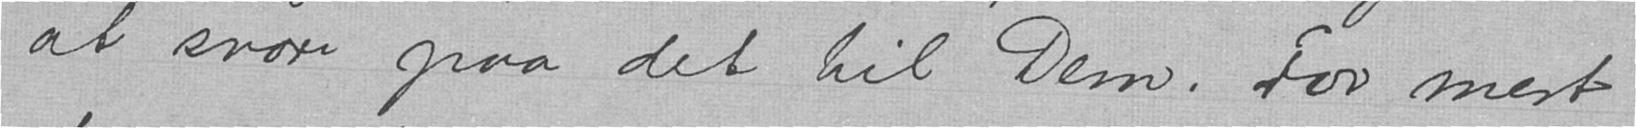at svare paa det til Dem. For mest
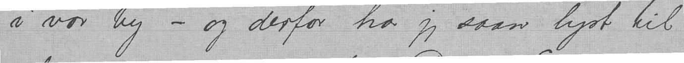i vor by - og derfor har jeg saan lyst til
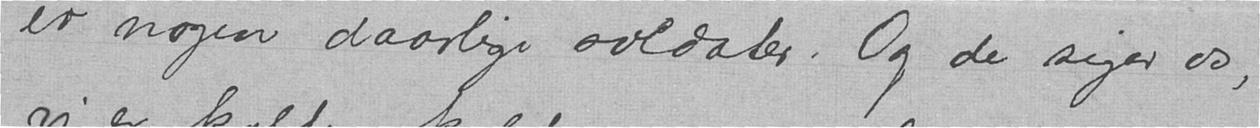er nogen daarlige soldater. Og de siger os,
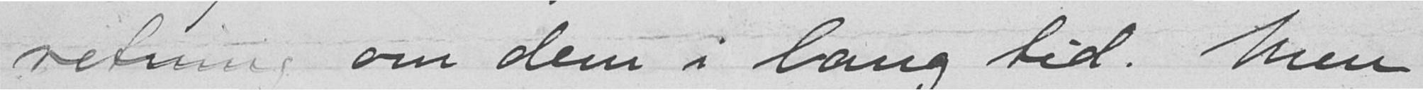retning om dem i lang tid. Men
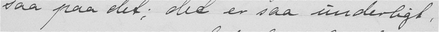saa paa det; det er saa underligt,
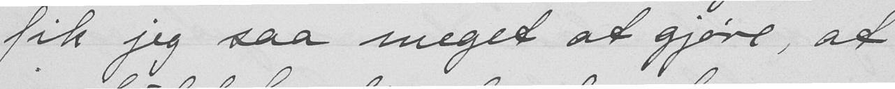fik jeg saa meget at gjøre, at
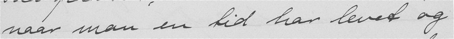naar man en tid har levet og
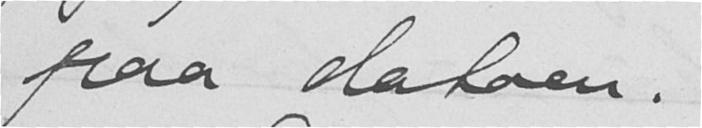paa datoen.
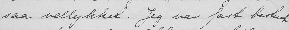saa vellykket. Jeg var fast bestemt
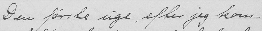Den første uge, efter jeg kom
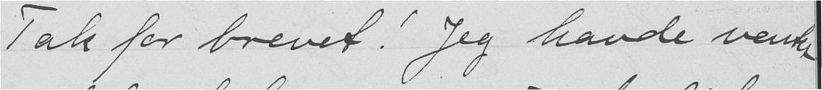Tak for brevet! Jeg havde ventet
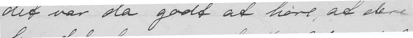det var da godt at høre, at dere
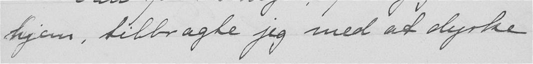hjem, tilbragte jeg med at dyrke
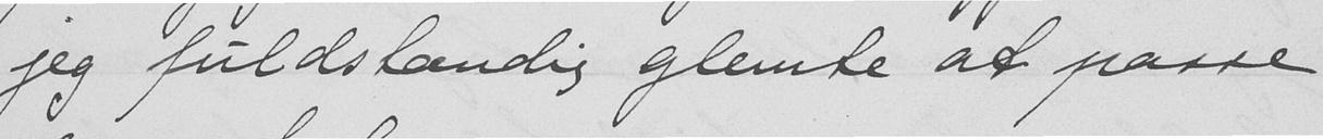jeg fuldstændig glemte at passe
